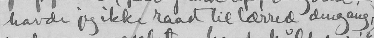havde jeg ikke raad til lærred dengang,)
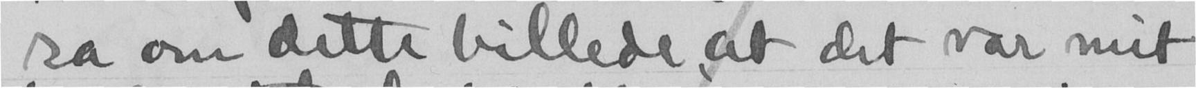sa om dette billede, at det var mit
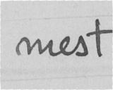mest
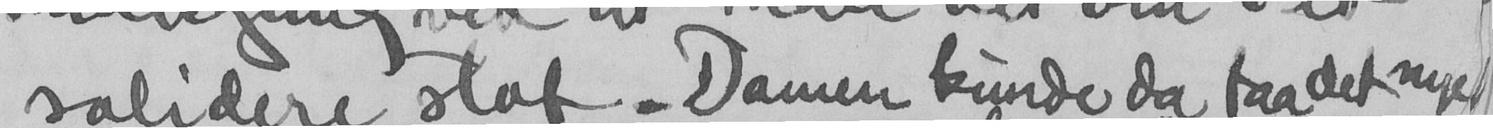solidere stof. Damen kunde da faa det nye
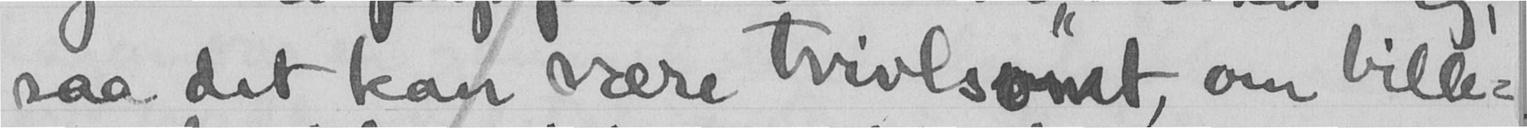saa det kan være tvivlsomt, om bille-
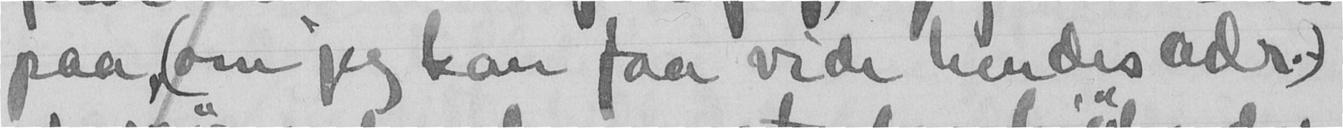paa, (om jeg kan faa vide uendes adr.)
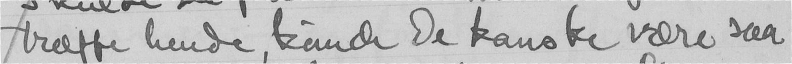træffe hende, kunde De kanske være saa
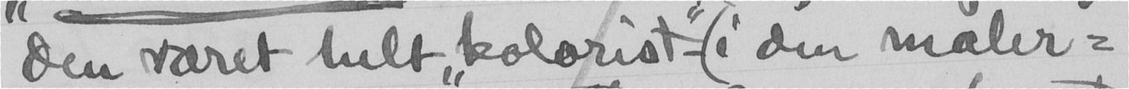den været helt "kolorist" - (i den maler-
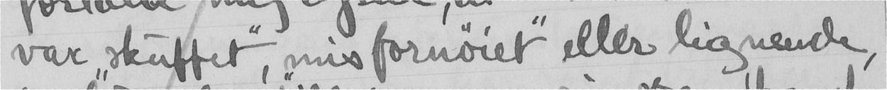var "skuffet", "misfornøiet" eller lignende,
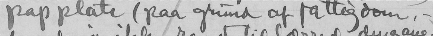papplate (paa grund af fattigdom, -
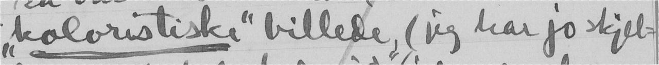"koloristiske" billede, (jeg har jo skjel-
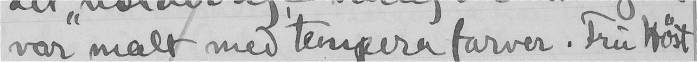var malt med tempera farver. Fru Høst
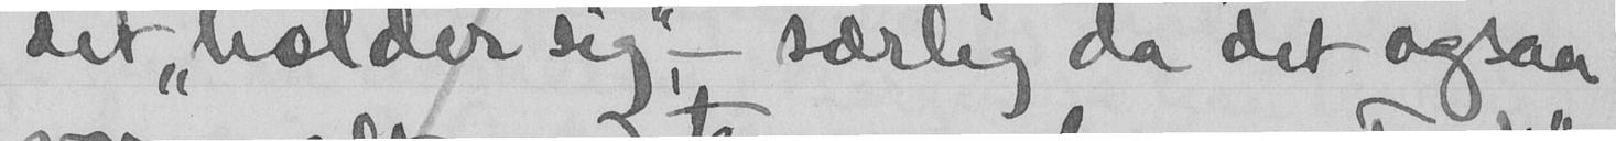det "holder sig",- særlig da det ogsaa
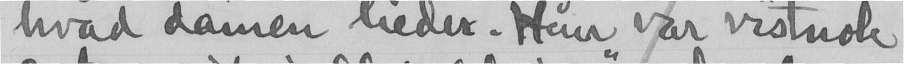hvad damen heder. Hun var vistnok
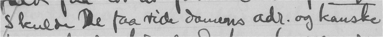Skulde de faa vide damens adr. og kanske
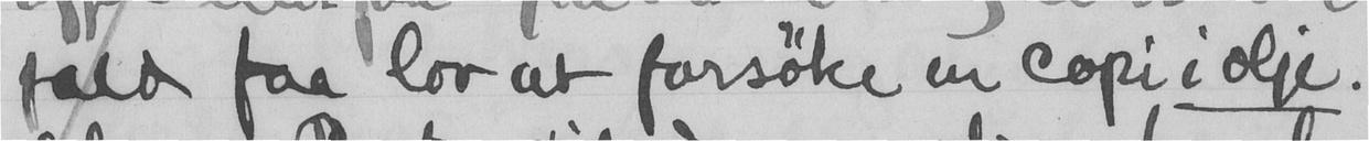fald faa lov at forsøke en copi i olje.
